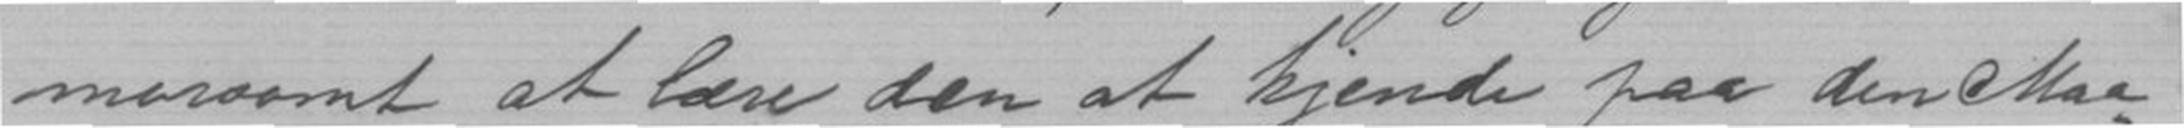morsomt at lære den at kjende paa den Maa-
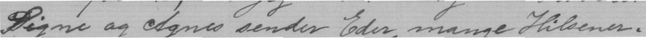Signe og Agnes sender Eder menge Hilsener.
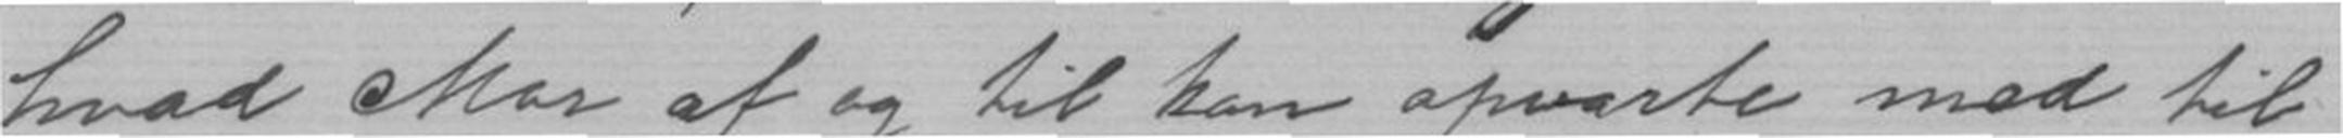hvad Mor af og til kan opvarte med til
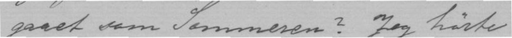gaaet som Sommeren? Jeg hørte
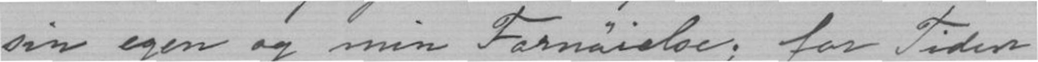sin egen og min Fornøielse; for Tiden
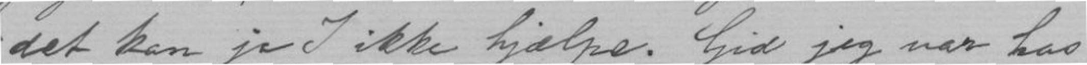det kan jo ikke hjælpe. Did jeg var hos
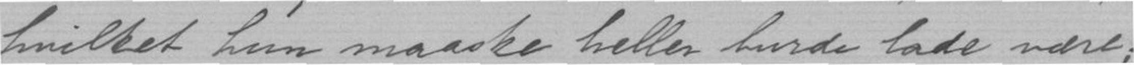hvilket hun maaske heller burde lade være;
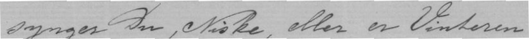synger Du, Niske, eller er Vinteren
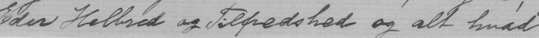Eder Helbred og Tilfredshed og alt hvad
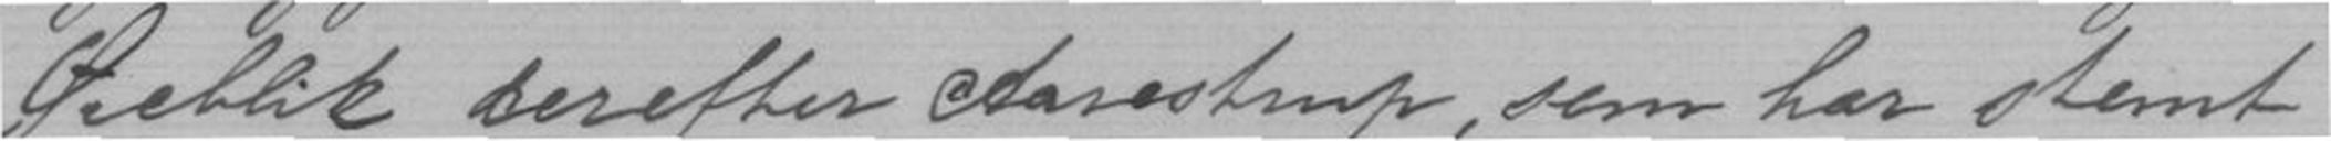Øieblik derefter Aarestruo, som har stemt
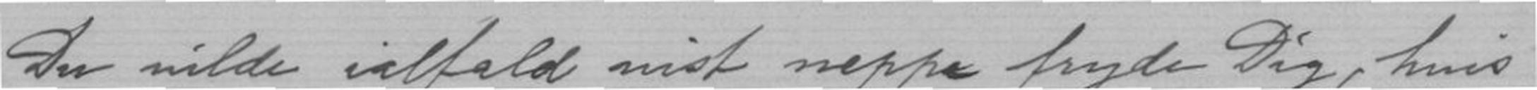Du vilde ialfald vist neppe fryde Dig, hvis
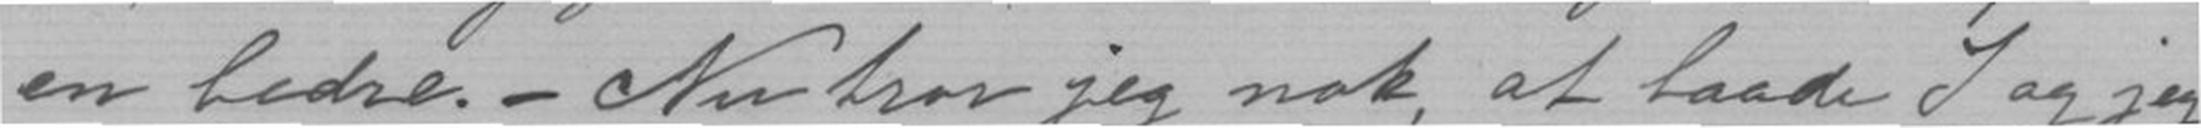en bedre. - Nu tror jeg nok, at baade I og jeg
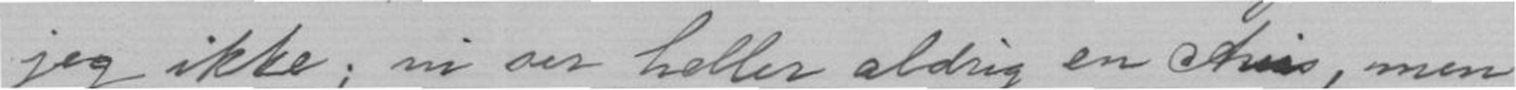jeg ikke; vi ser heller aldrig en Aries, men
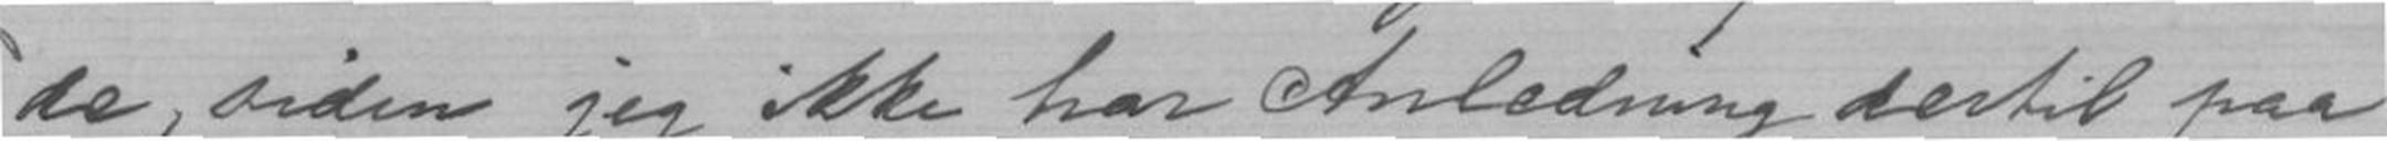de, siden jeg ikke har Anledning dertil paa
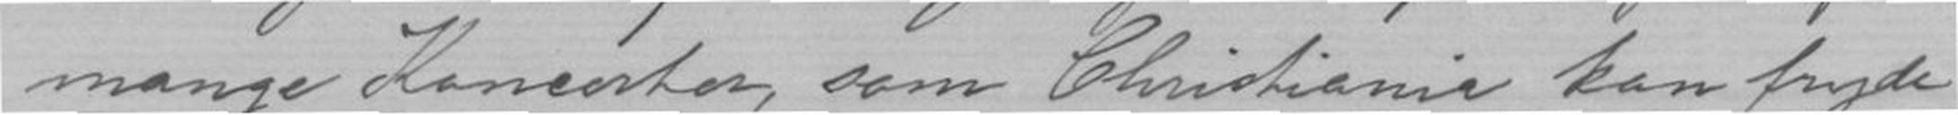mange Konserter, som Christiania kan fryde
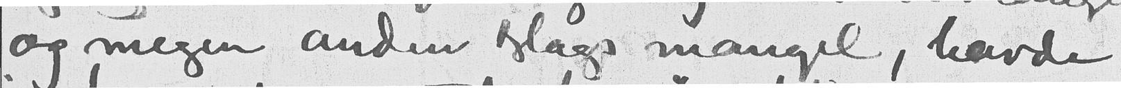og megen anden slags mangel, havde
